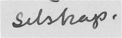selskap.
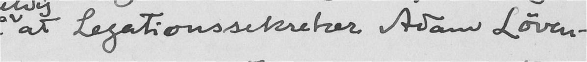at Legationssekretær Adam Løven-
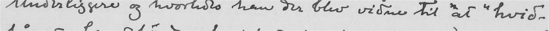"Underliggere" og hvorledes han der blev vidne til at "hvid-
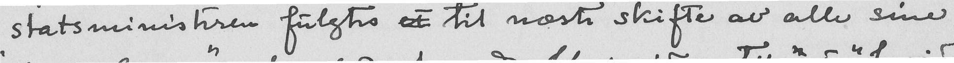statsministren fulgtes et til næste skifte av alle sine
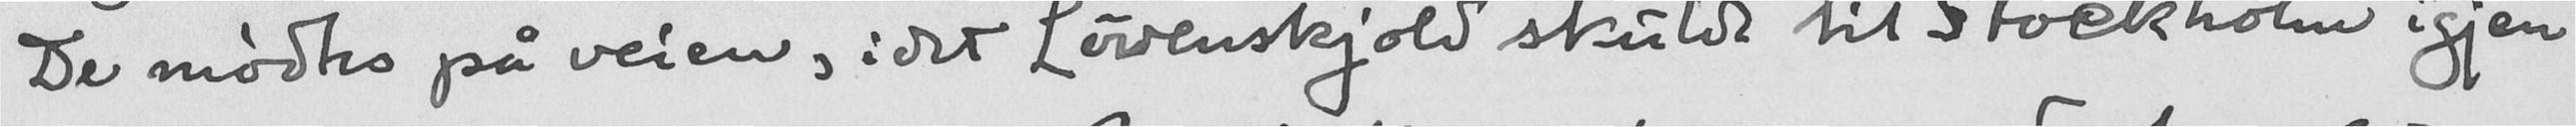De mødtes på veien, i det Løvenskjold skulde til Stockholm igjen
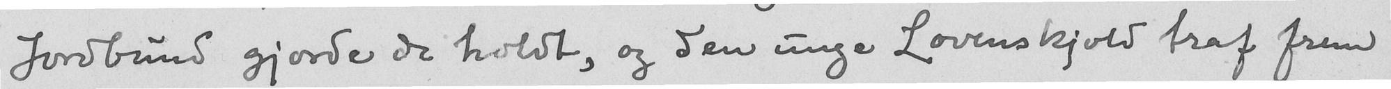Jordbund gjorde de holdt, og den unge Lovenskjold traf frem
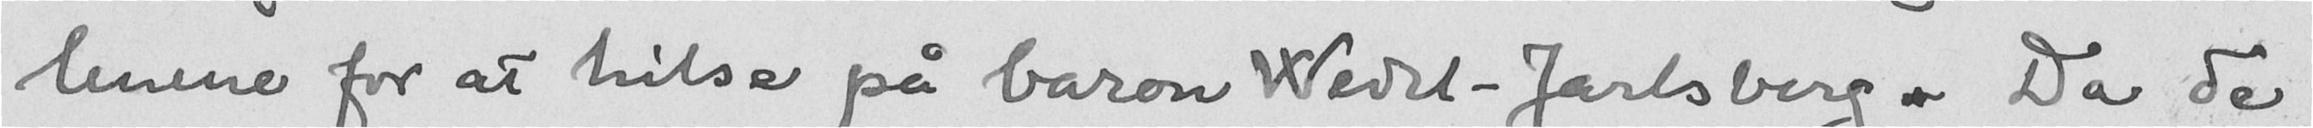lenene for at hilse på baron Wedel-Jarlsberg. Da de
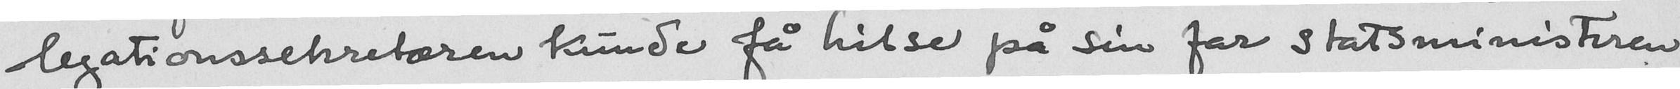legationssekretæren kunde få hilse på sin far statsministeren.
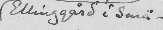Ellinggård i Små-
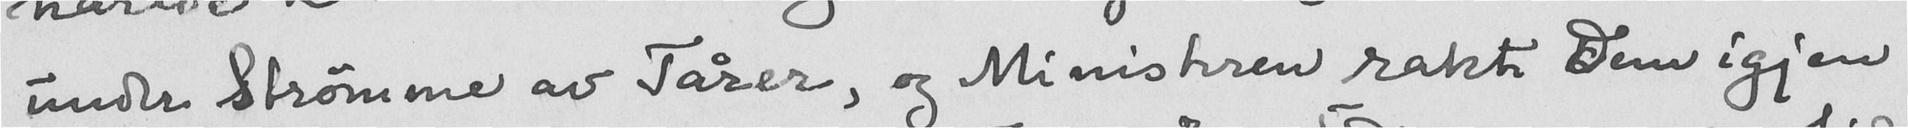under strømme av Tårer, og Ministeren rakte Dem igjen
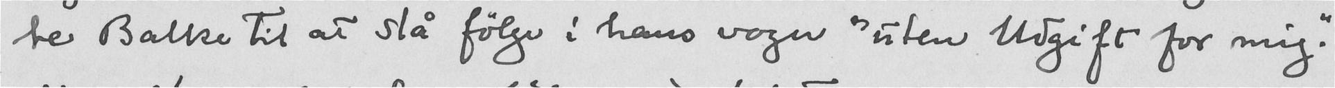te Balke til at slå følge i hans vogn "uten Udgift for mig."
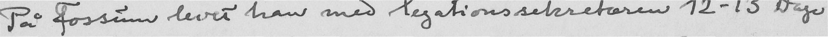På Fossum levet han med legationssekretæren 12-13 Dage
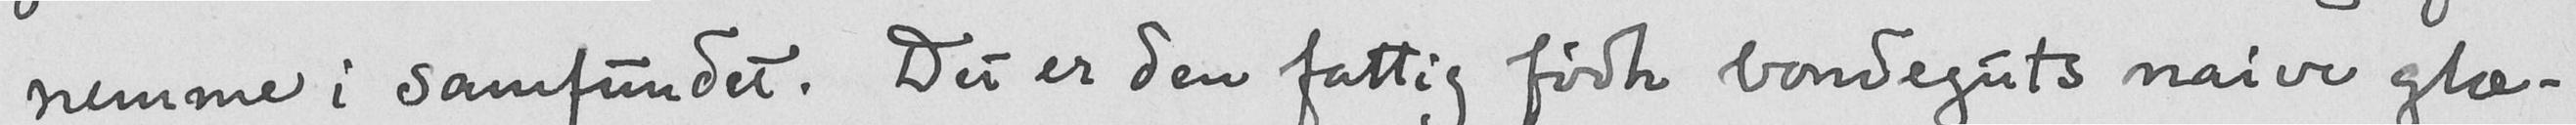nemme i samfundet. Det er den fattig fødte bondeguts naive glæ-
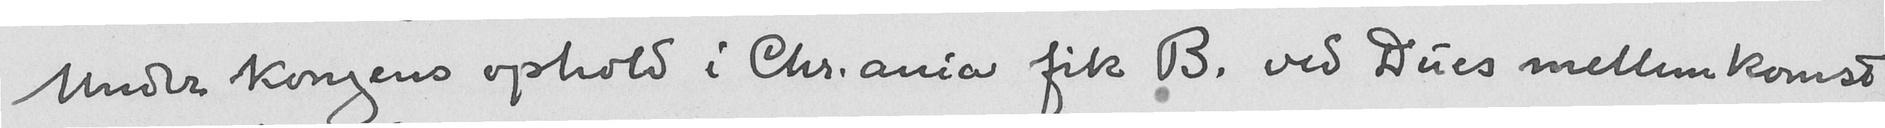Under kongens ophold i Chr.ania fik B. ved Dues mellemkomst
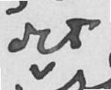det
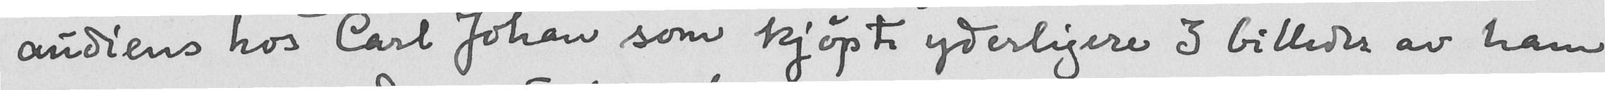audiens hos Carl Johan som kjøpte yderligere 3 billeder av ham
